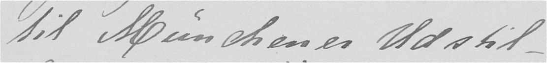til Münchenes Udstil-
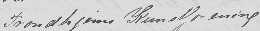Trondhjems Kunstforening.
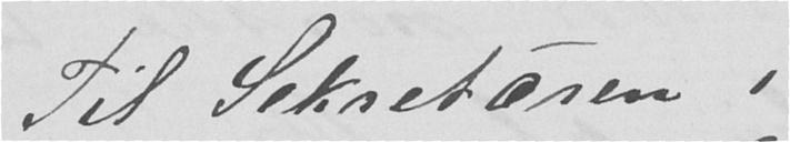Til Sekretæren i
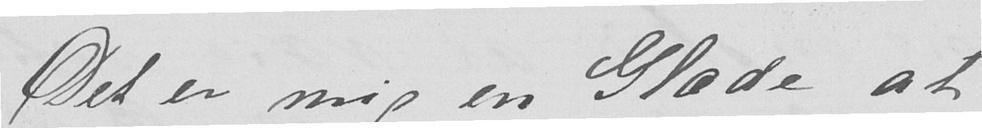Det er mig en Glæde at
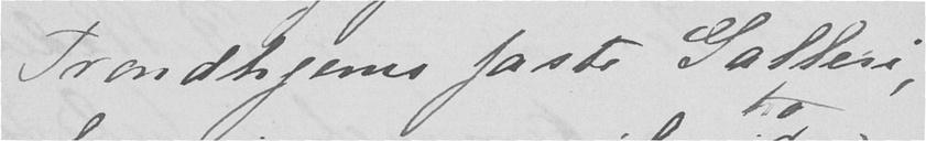Trondhjems faste Galleri,
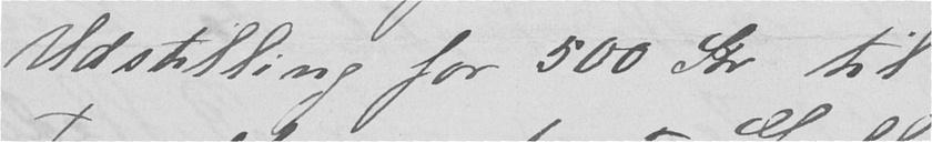Udstilling for 500 Kr til
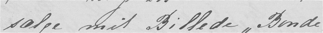sælge mit Billede "Bonde-
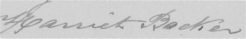Harriet Backer
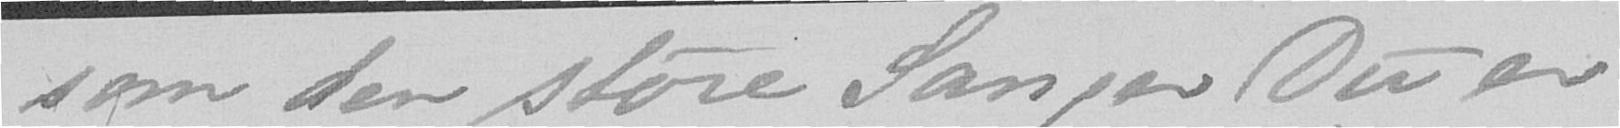som den store Sanger Du er
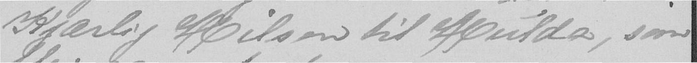Kjærlig Hilsen til Hulda, som
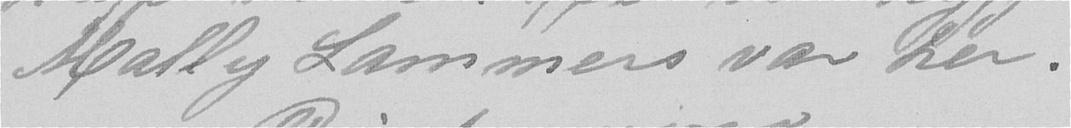Mally Lammers var her.
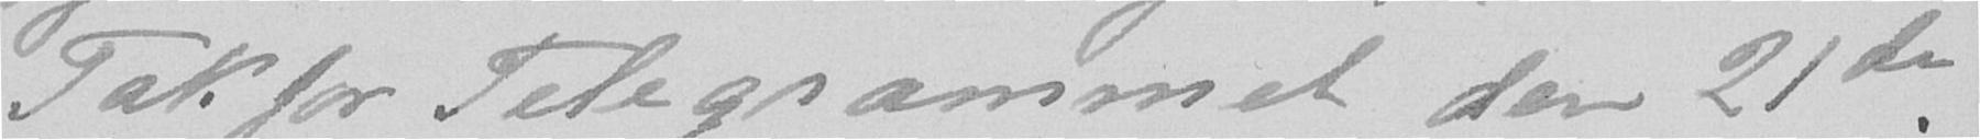Tak for Telegrammet den 21de.
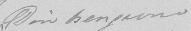Din hengivne
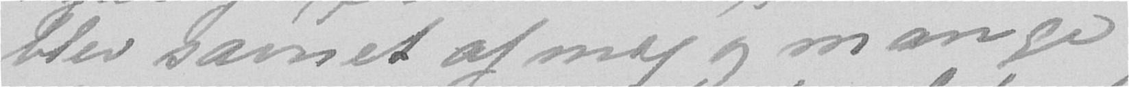blev savnet af mig og mange
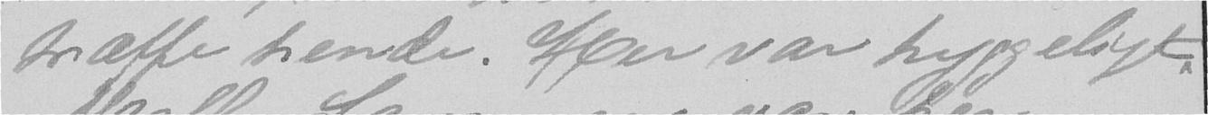tæffe hende. Her var hyggeligt.
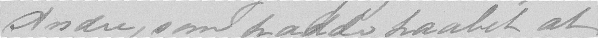Andre, som hadde haabet at
